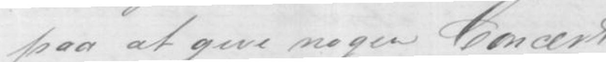paa at give nogen Concert.
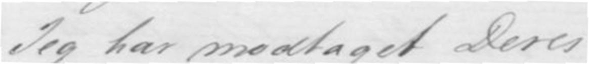Jeg har modtaget Deres
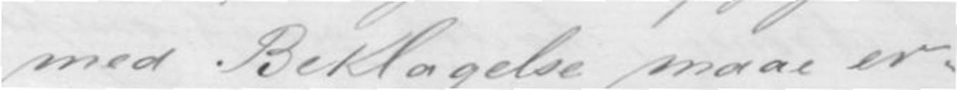med Beklagelse maae er-
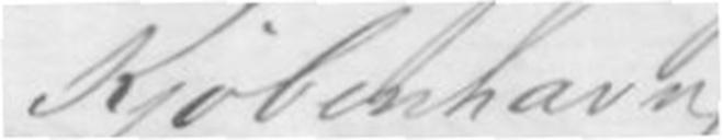Kjøbenhavn
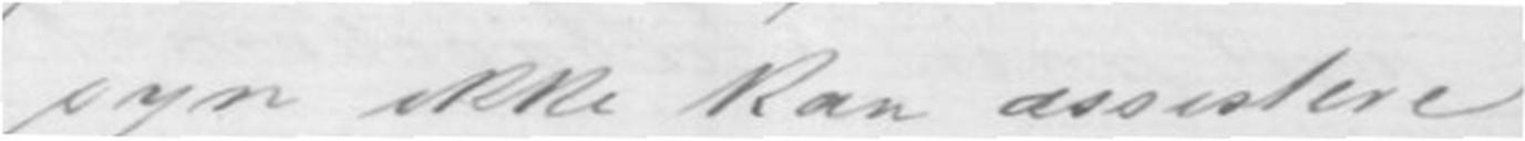syn ikke kan assistere
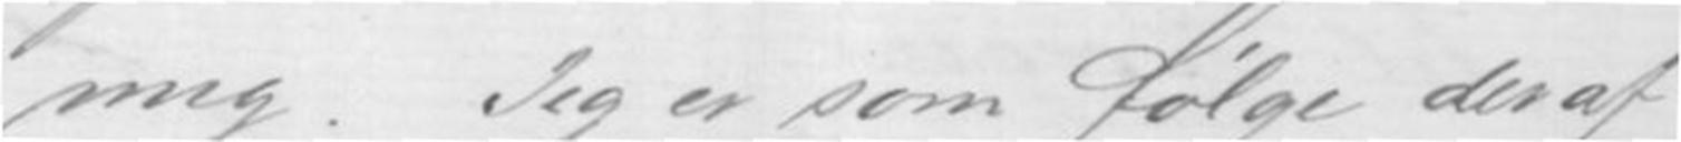mig. Jeg er som følge der af
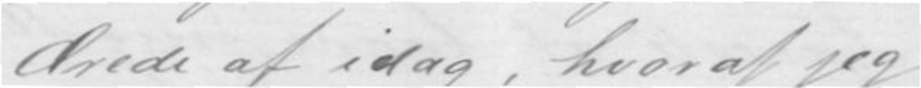Ærede af idag, hvoraf jeg
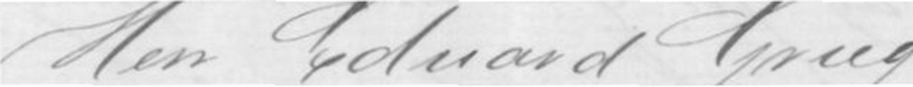Herr Edvard Grieg
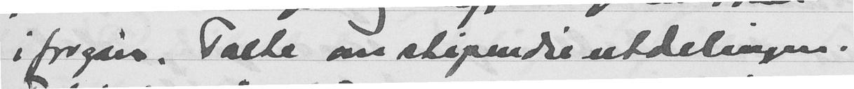iforgårs. Talte om stipendieutdelingen.
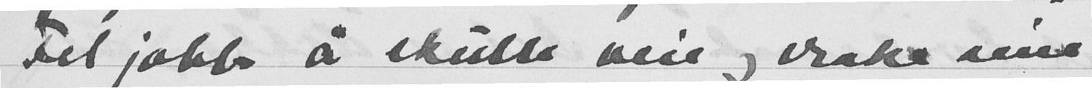Fel jobb å skulle veie og vrake mine
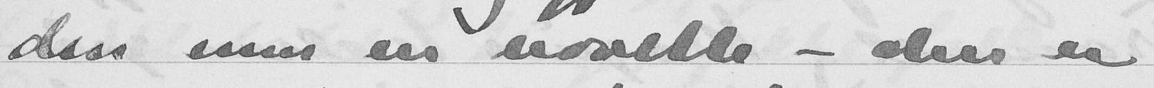den enn en novelle - den er
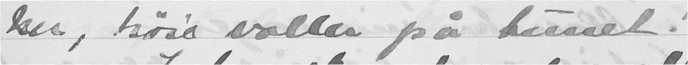her, høie voller på tunet!
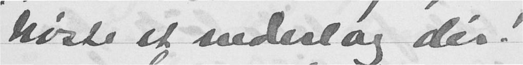høste et nederlag dér!
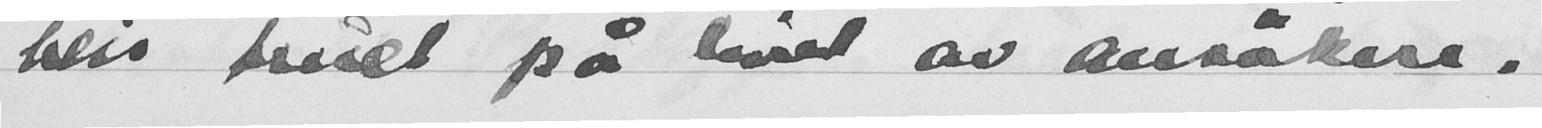blir truet på liver av ansøkere.
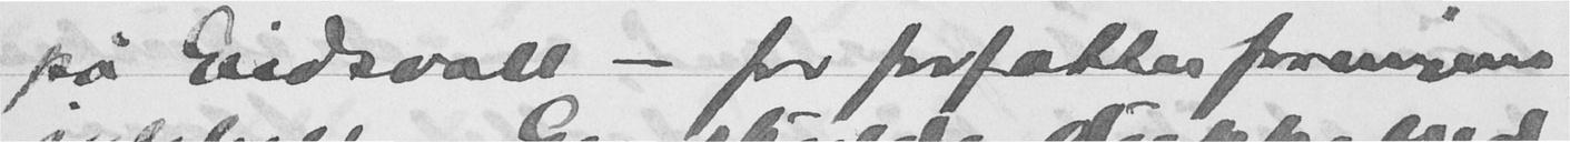på Eidsvoll - for forfatterforeningens
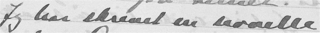Jeg har skrevet en novelle
